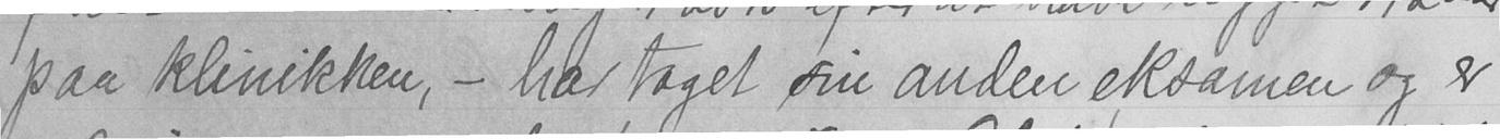paa klinikken, - har taget sin anden eksamen og er
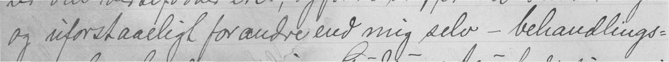og uforstaaeligt for andre end mig selv - behandlings-
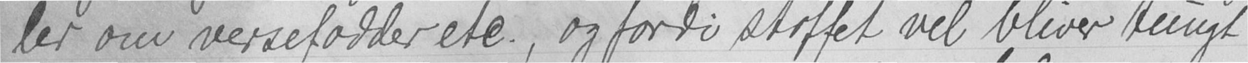ler om versefødder etc., og fordi stoffet vel bliver tungt
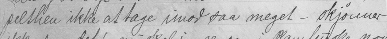pelthen ikke at tage imod saa meget - skjønner
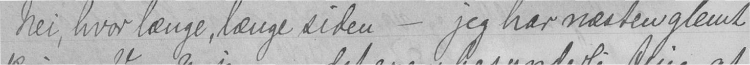Nei, hvor længe, længe siden - jeg har næsten glemt
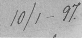10/1 - 97.
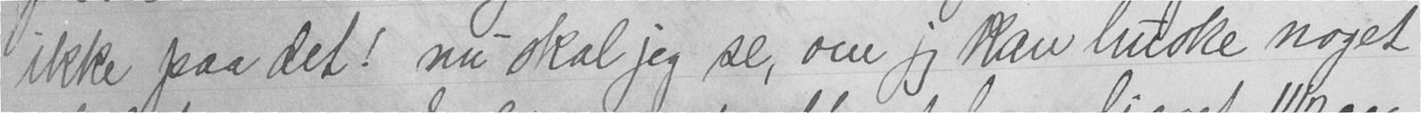ikke paa det! nu skal jeg se, om jeg kan huske noget
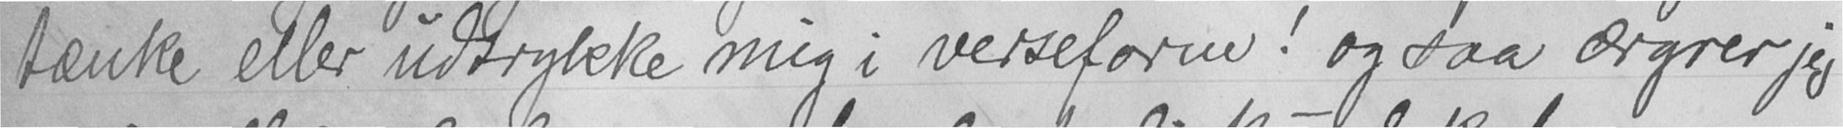tænke eller udtrykke mig i verseform! og saa ærgrer jeg
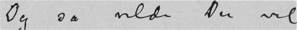Og så vilde du vel
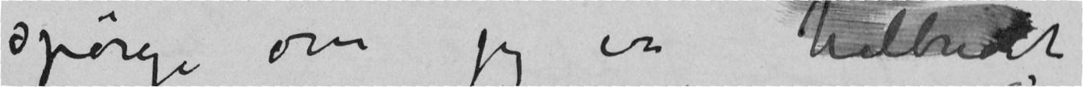spørge om jeg er helbredet
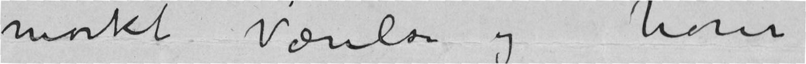mørkt Værelse og hører
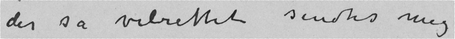der så velrettet sendtes mig
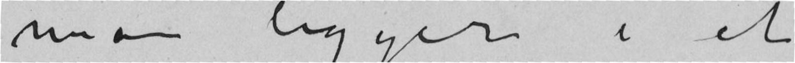man ligger i et
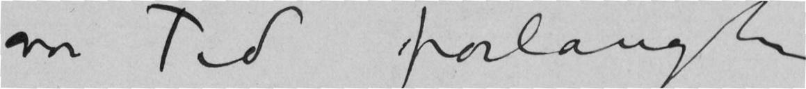vor Tid forlangte
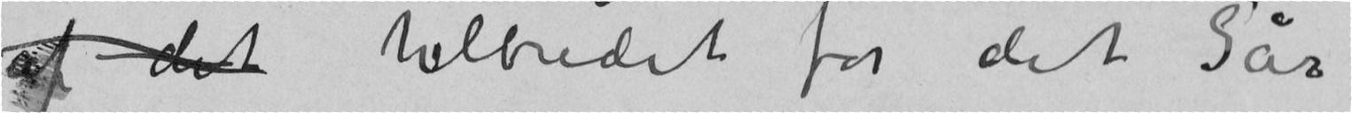af det helbredet for det Sår
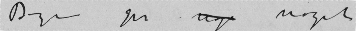Bogen gir noget
